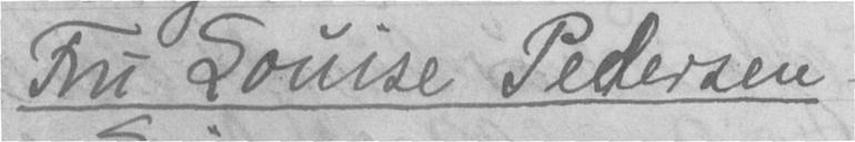Fru Louise Pedersen
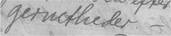gernetheder. -
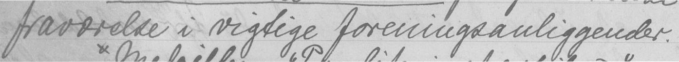fraværelse i vigtige foreningsanliggender.
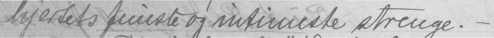hjertets fineste og intimeste strenge. -
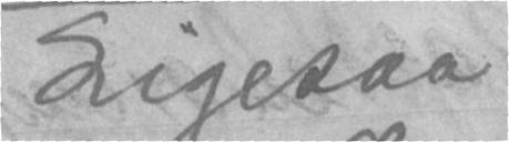Ligesaa
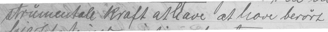strumentale kraft at have at hove berørt
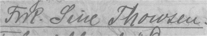Frk. Sine Thowsen.
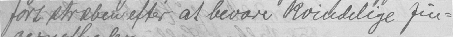ført stræben efter at bevare kvindelige fin-
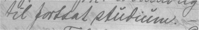til fortsat studium. -
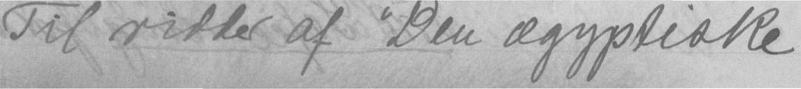Til ridder af "Den ægyptiske
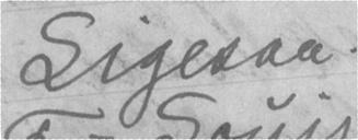Ligesaa:
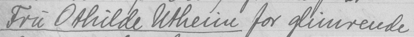Fru Othilde Utheine for glimrende
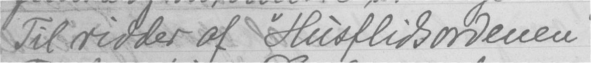Til ridder af "Husflidsordenen"
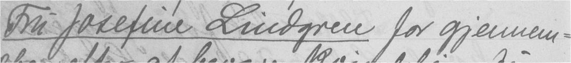Fru Josefine Lindgren for gjennem-
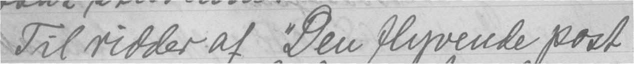Til ridder af "Den flyvende post"
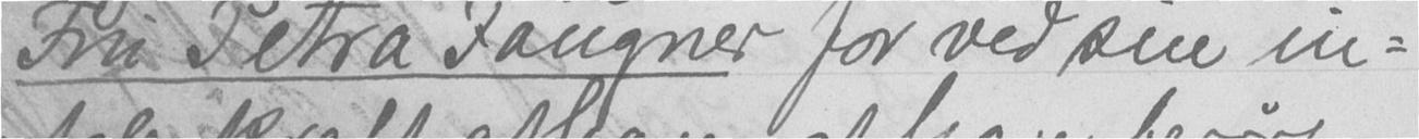Fru Petra Faugner for ved sin in-
